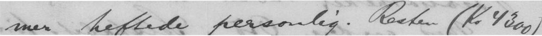mer heftede personlig. Resten (Kr 4300)
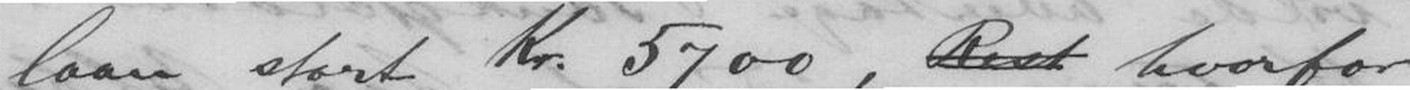laan stort Kr. 5700, Rest hvorfor
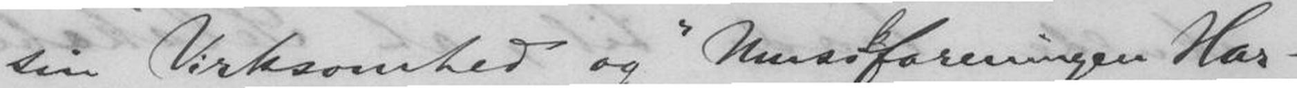sin Virksomhed og "Musikforeningen Har-
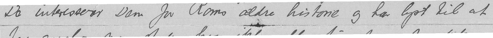De interesserer Dem for Roms ældre historie og har lyst til at
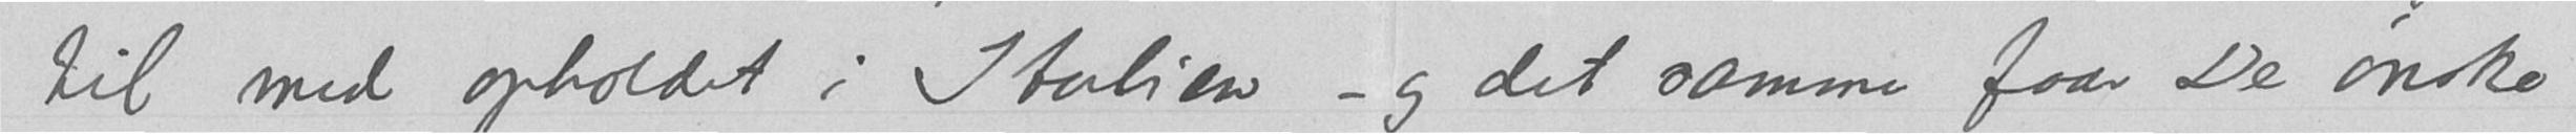til med opholdet i Italien – og det samme faar De ønske
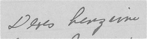Deres hengivne
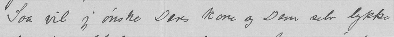Saa vil jeg ønske Deres kone og Dem selv lykke
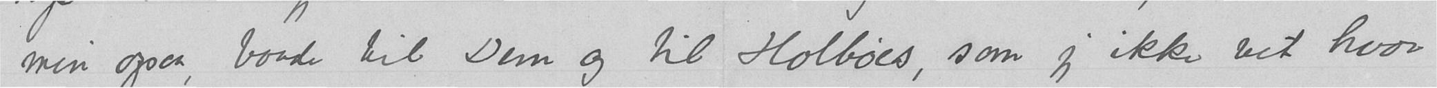min ogsaa, baade til Dem og til Holbøes, som jeg ikke vet hvor
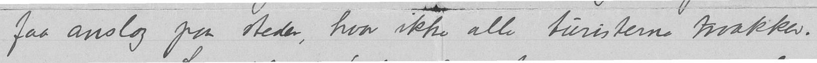faa anslag paa steder, hvor ikke alle turisterne traakker.
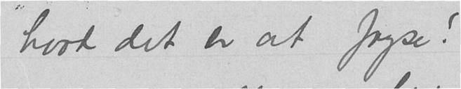hvad det er at fryse!
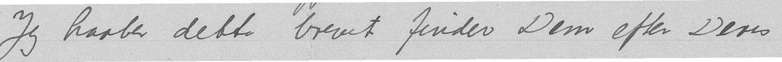Jeg haaber dette brevet finder Dem efter Deres
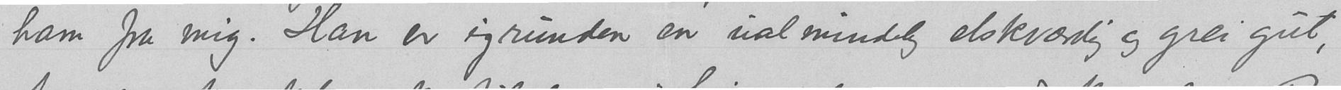ham fra mig. Han er igrunden en ualmindelig elskværdig og grei gut,
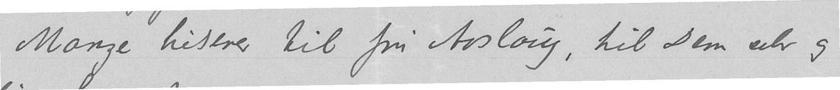Mange hilsener til fru Aaslaug, til Dem selv og
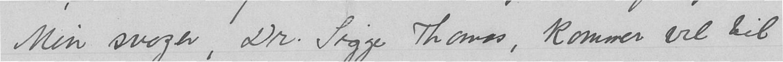Min svoger, Dr. Sigge Thomas, kommer vel til
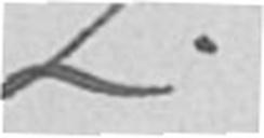2.
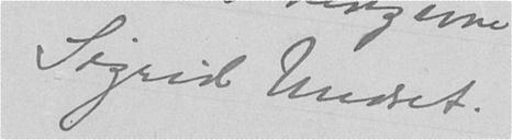Sigrid Undset.
